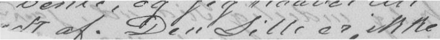af. Den Lille er ikke
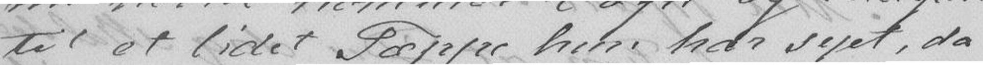til et lidet Teppe hun har syet, da
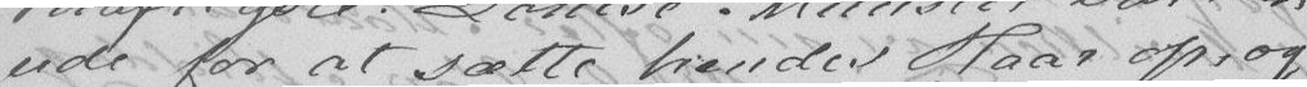ude for at sætte hendes Haar op, og
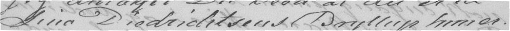Lina Diedrichtsens Bryllup hun er.
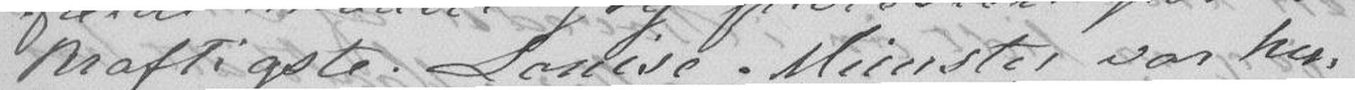kraftigste. Louise Münster var her
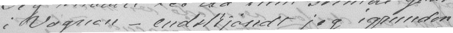i Vognen - endskjøndt jeg igrunden
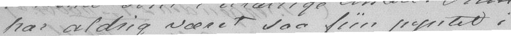har aldrig været saa fiin pyntet i
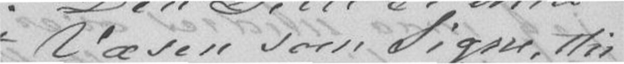Væsen som Signe, thi
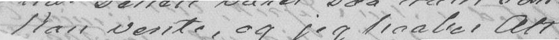kan vente, og jeg haaber Alt
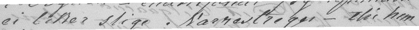ei liker slige Narrestreger - thi hun
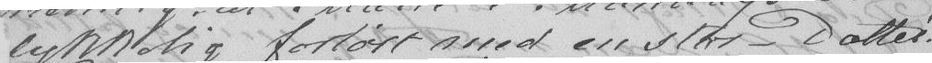lykkelig forløst med en stor - Datter!
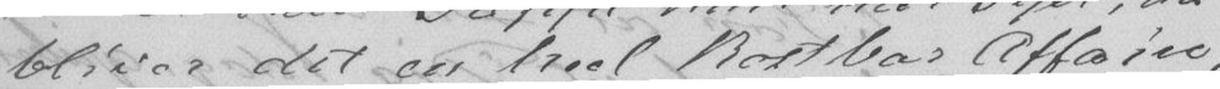bliver det en heel kosbar Affaire,
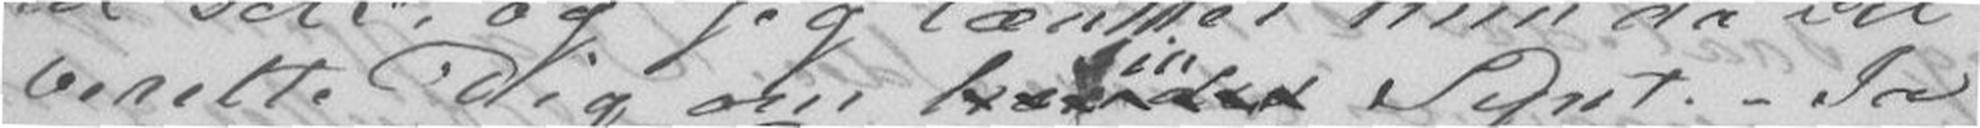berette Dig om hendes sin Pynt. Jo
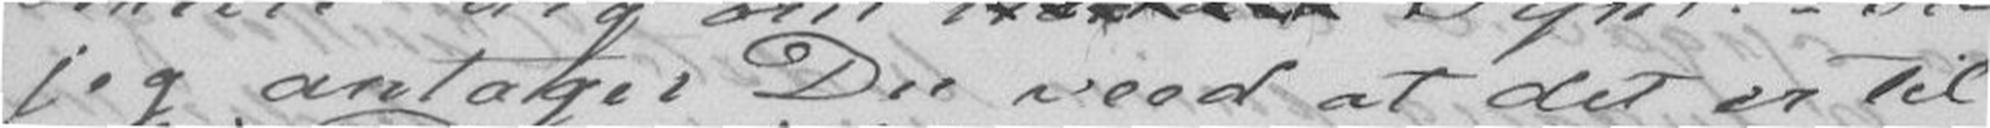jeg antager Du veed at det er til
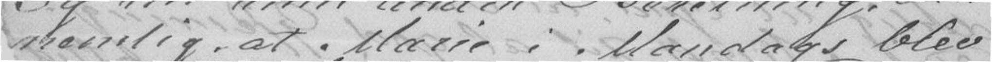næmlig at Marie i Mandags blev
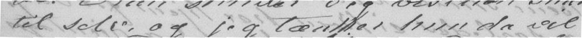til selv, og jeg tænker hun da vil
